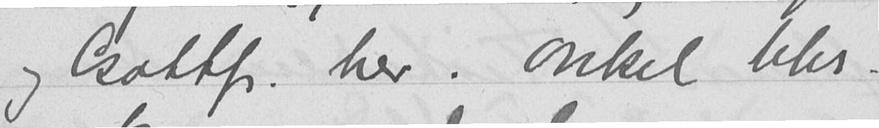og Gottfr. her. Onkel Chr.
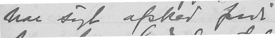har søgt afsked fordi
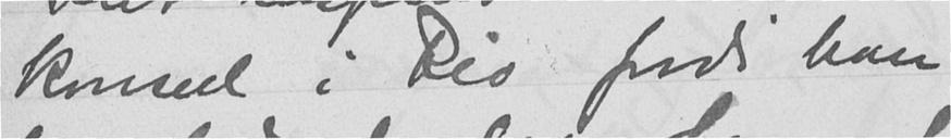konsul i Rio fordi han
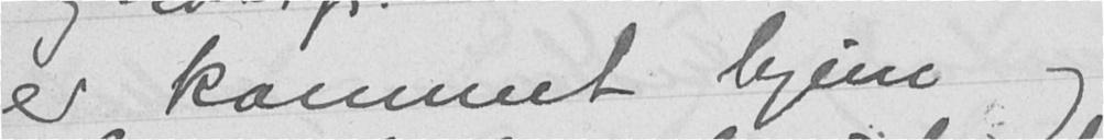er kommet hjem og
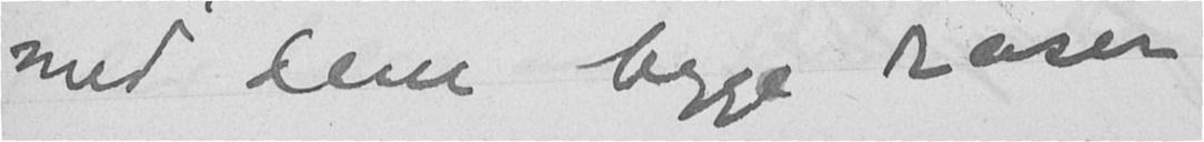med dem begge raser
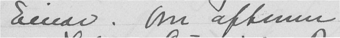Einar. Om aftenen
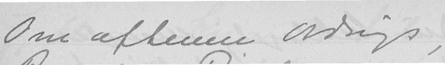Om aftenen Ordings,
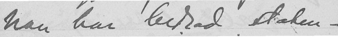han har bedrad staten -
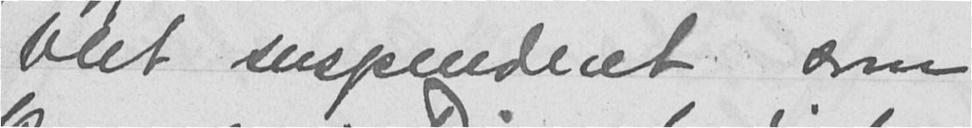blit suspenderet som
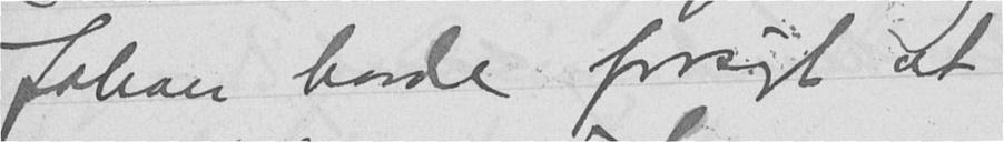Johan havde forsøgt at
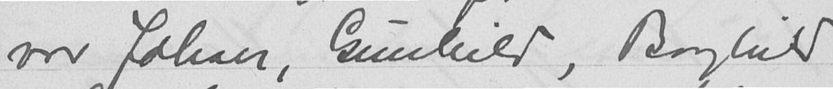var Johan, Gunhild, Borghild
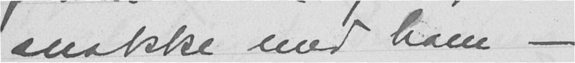snakke med ham -
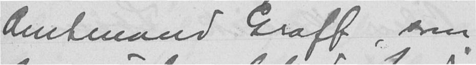Amtmand Graff, som
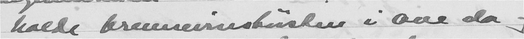holde brennevinstørsten i veie da og
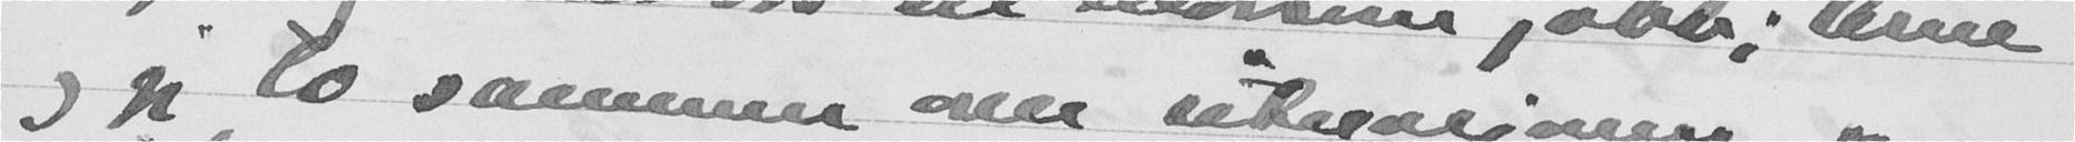og jeg lo sammen over situasjonen, men
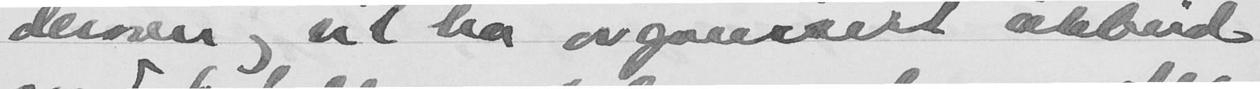derover og vil ha organisert arbeid
